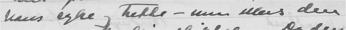hans syke og trette - men ellers den
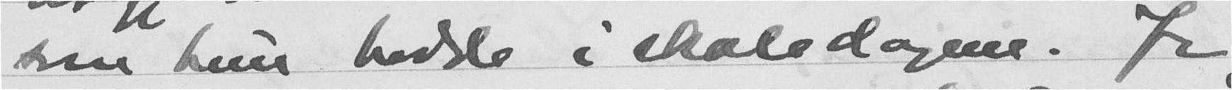som hun hadde i skoledagene. fe
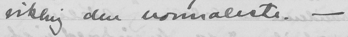vikling den normaleste. -
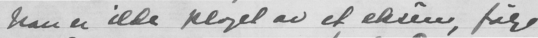han er ille plaget av et eksem, følge
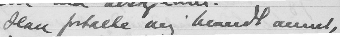Han fortalte mig blandt annet,
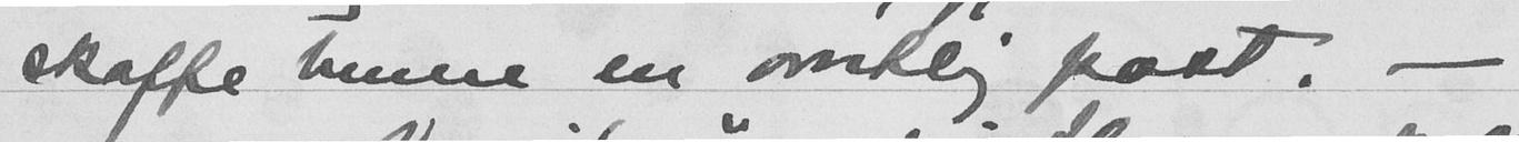skaffe henne en orntlig post. -
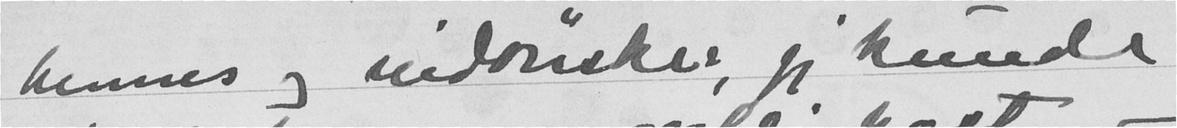hennes og indønsker, jeg kunde
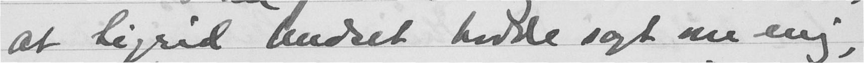at Sigrid Undset hadde sagt om mig,
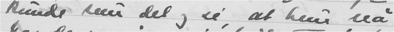kunde len det og si, at hun nå
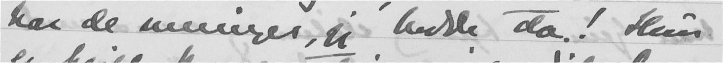har de meningene, jeg hadde da! Hun
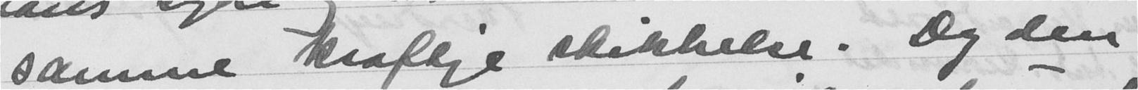samme kraftige skikkelse. Og den
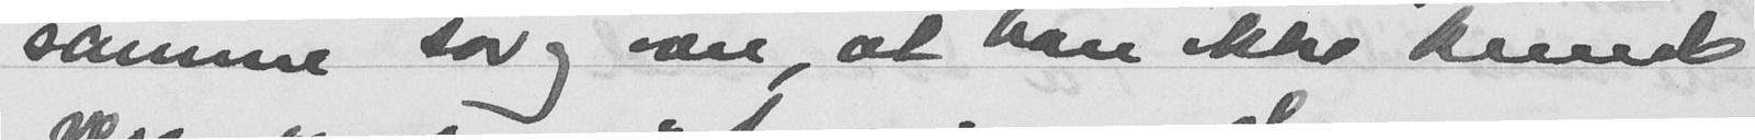samme sorg over at han ikke kunde
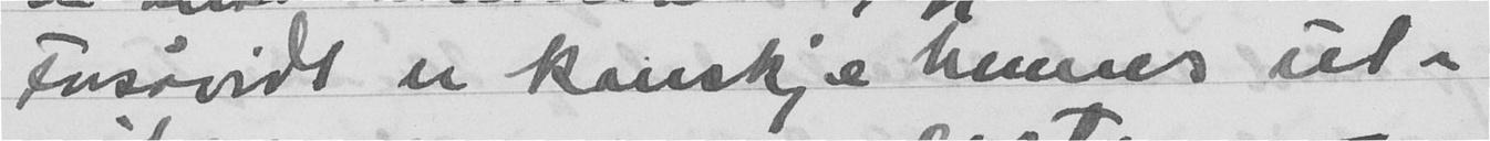Forsåvidt er kanskje hennes ut-
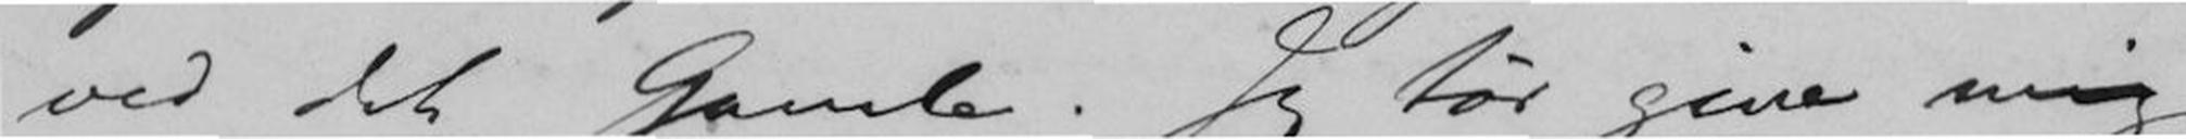ved det Gamle. Jeg tør give mig
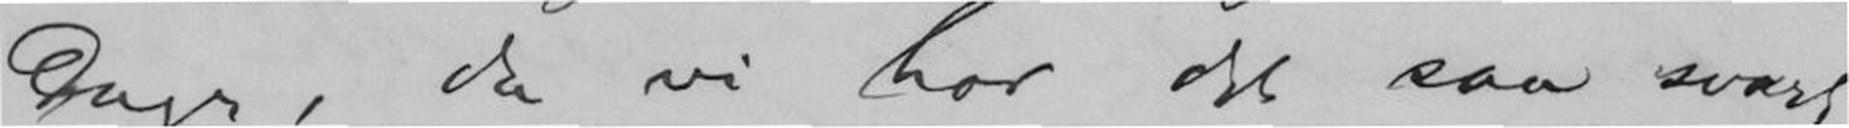Dage, da vi har det saa svart
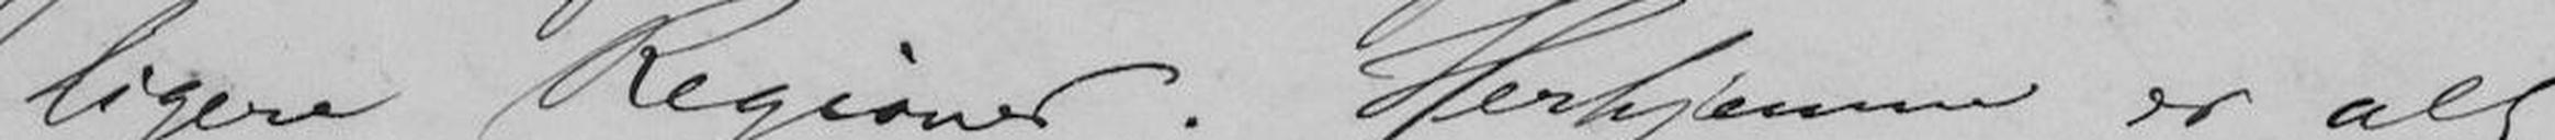ligere Regioner. Herhjemme er alt
